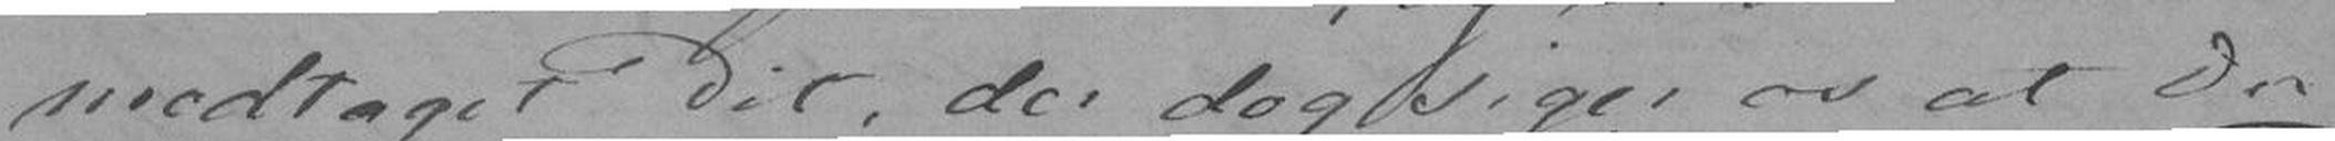modtaget Dit, der dog siger os at Du
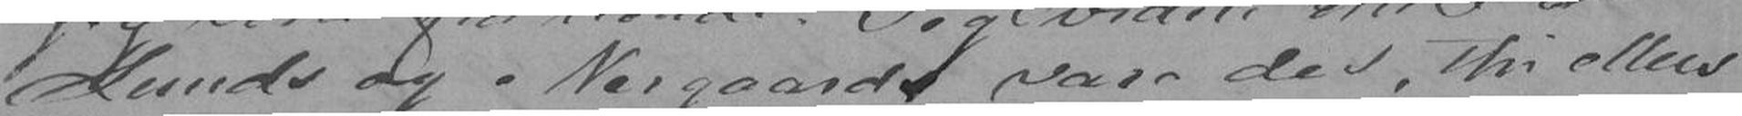Lunds og Nergaads vare der, thi ellers
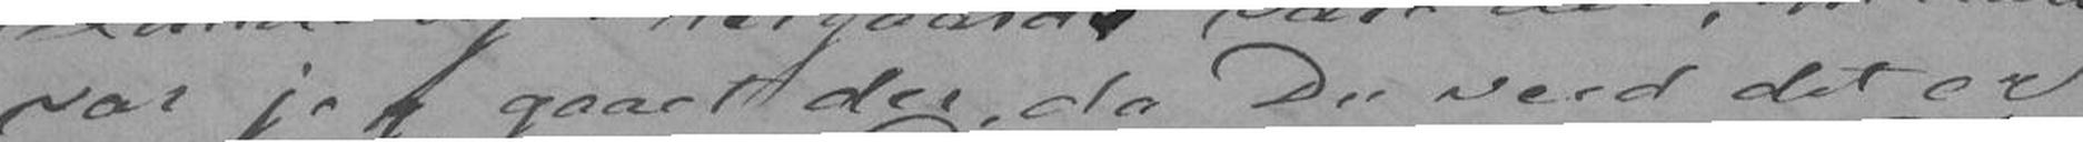var jeg gaaet der, da Du veed det er
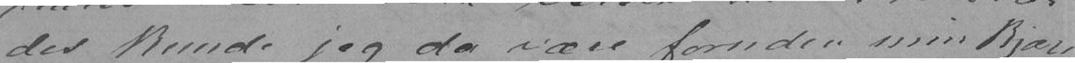des kunde jeg da være foruden min kjære,
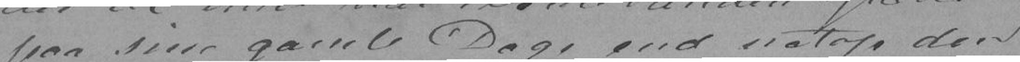paa sine gamle Dage end netop den
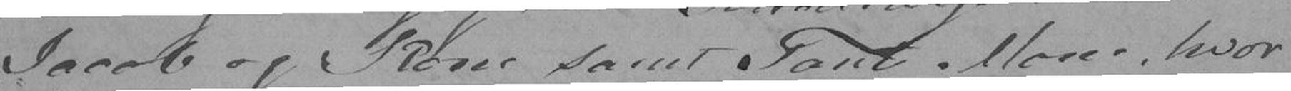Jacob og Kone samt Tante Mone, hvor
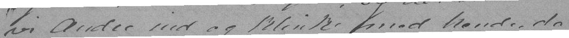vi Andre ind og klinke med hende, da
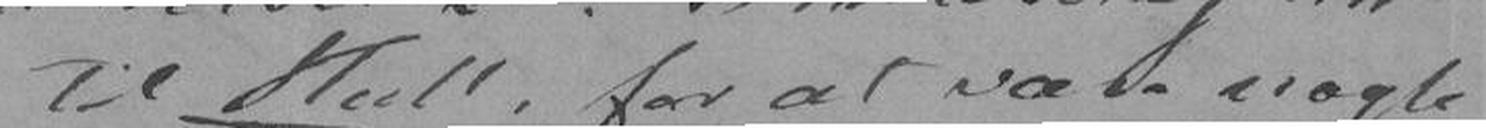til Hull, for at være nogle
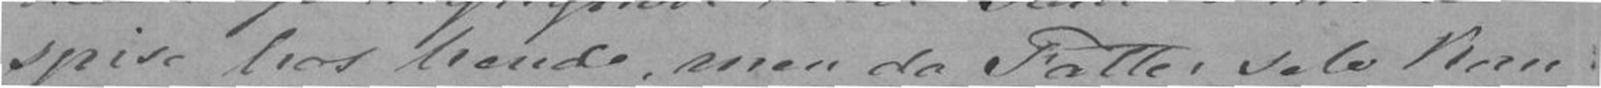spise hos hende, men da Fatter selv kom
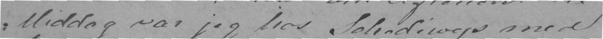Middag var jeg hos Schediwys med
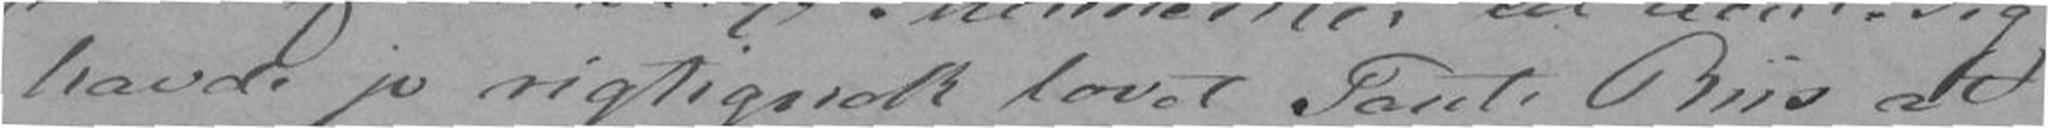havde jo rigtignok lovet Tante Riis at
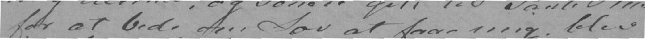for at bede om Lov at faae mig, blev
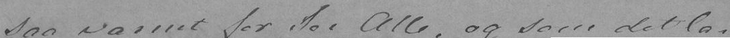saa varmt for Jer Alle, og som det la-
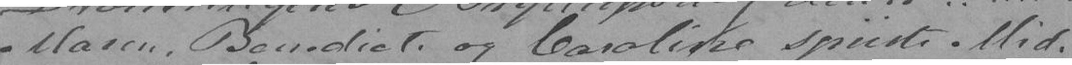Maren, Benedicte og Caroline spiiste Mid-
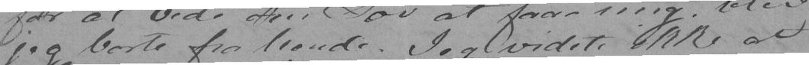jeg borte fra hende. Jeg vidste ikke at
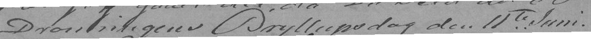Dronningens Bryllupsdag den 11te Juni.
